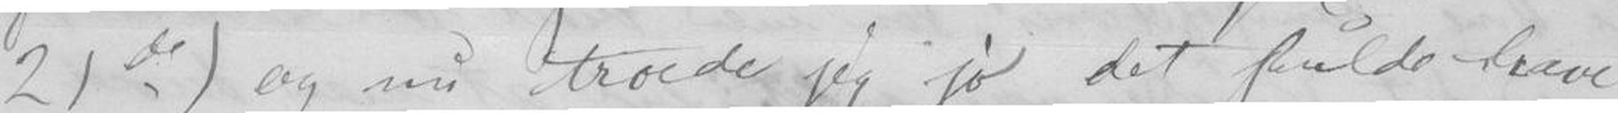21de) og nu troede jeg jo det skulde have
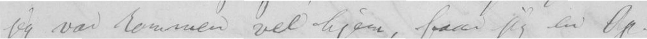jeg var kommen vel hjem, faar jeg en Op-
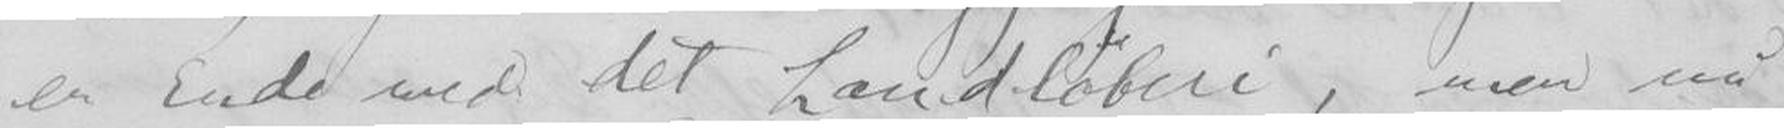en Ende med det Landløberi, men nu
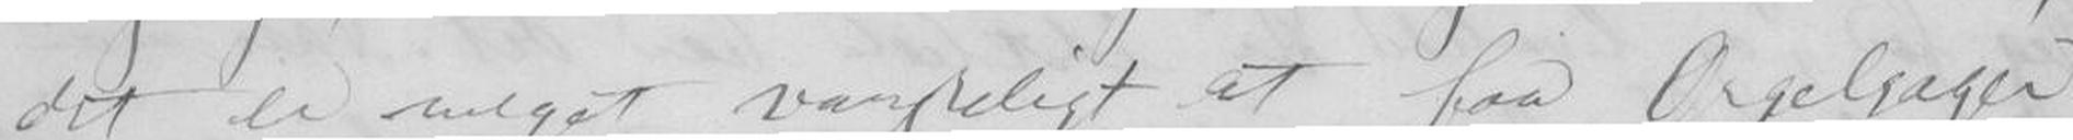det er meget vanskeligt at faa Orgelsager
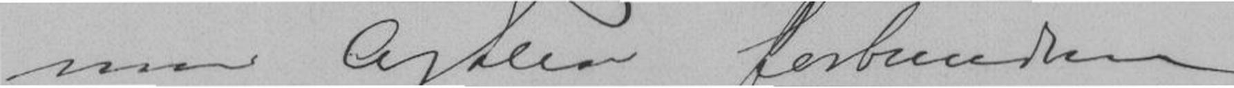med Agtelse forbundne
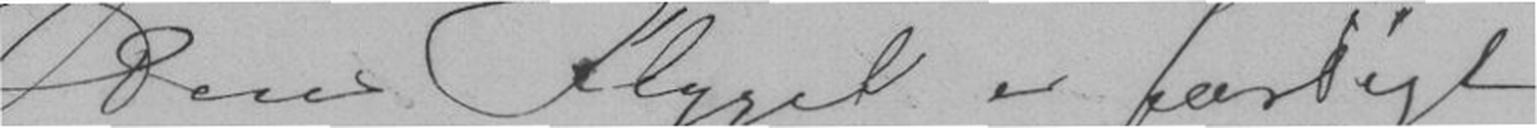Deres Flygel er færdigt.
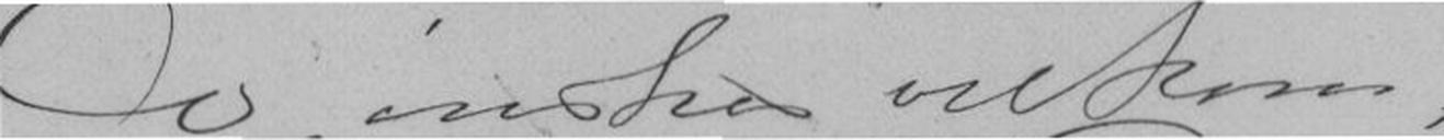De ønskes velkom-
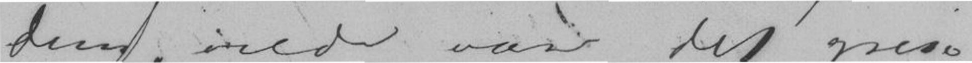dem, vilde være det greie-
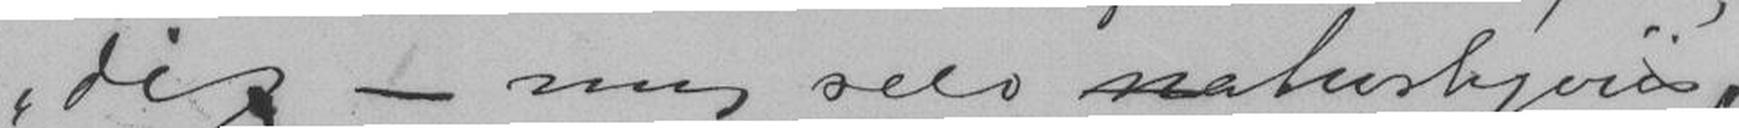=dig - mig selv naturligvis,
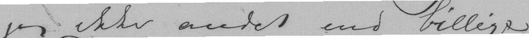jeg ikke andet end billige
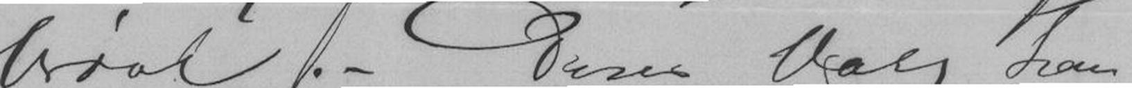Vrøvl. - Deres Valg kan
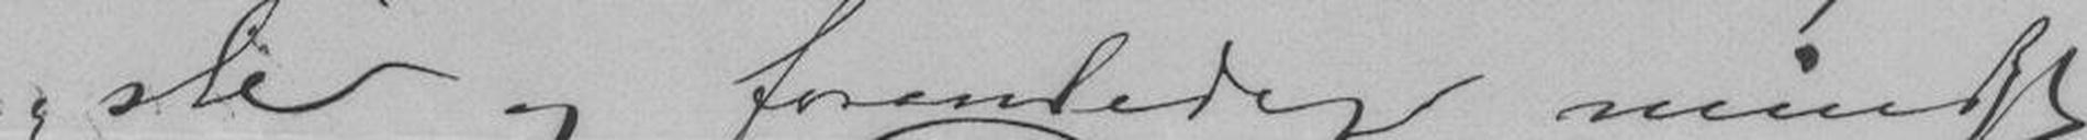ste og foranledige mindst
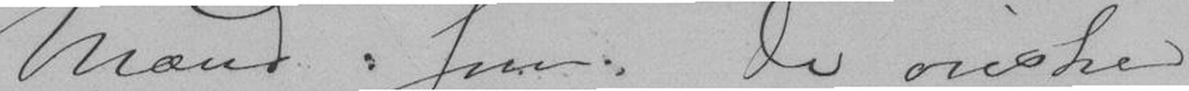Mænd som De ønsker
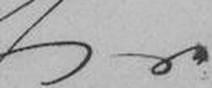Ps
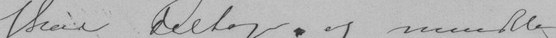skal deltage og mundtlig
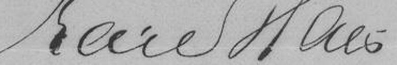Karl Hals
